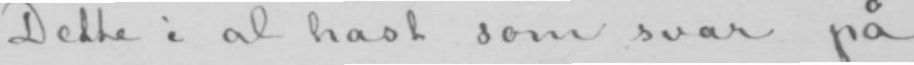Dette i al hast som svar på
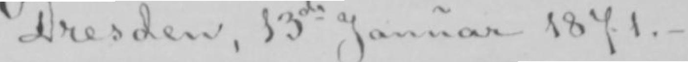Dresden, 13de Januar 1871.-
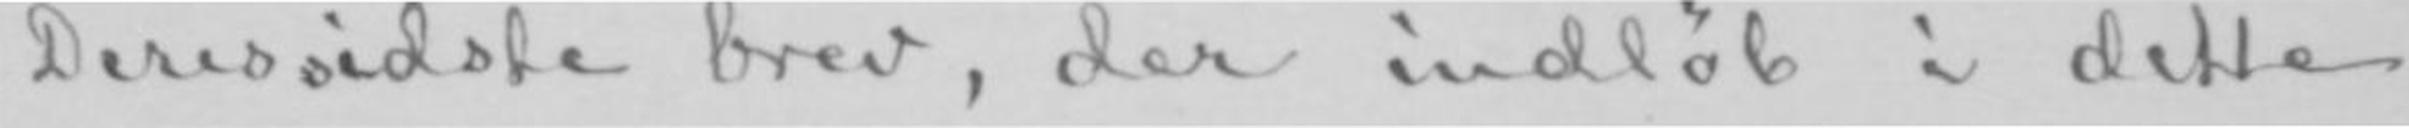Deres sidste brev, der indløb i dette
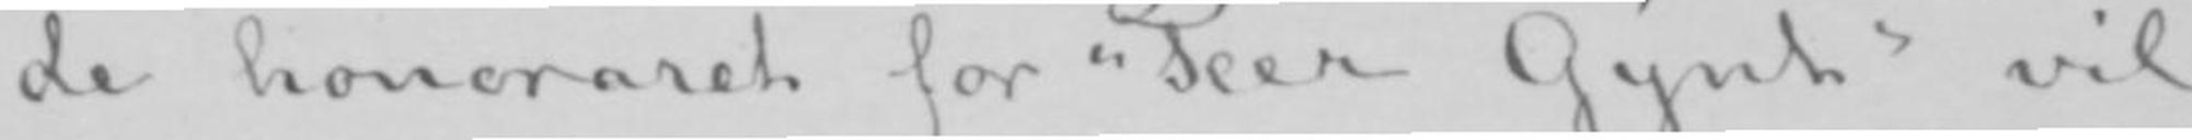de honoraret for «Peer Gynt» vil
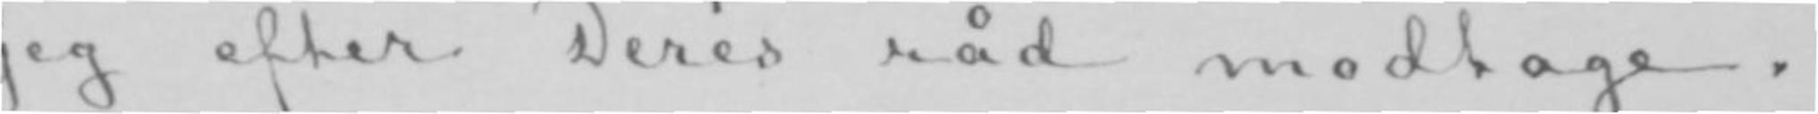jeg efter Deres råd modtage.
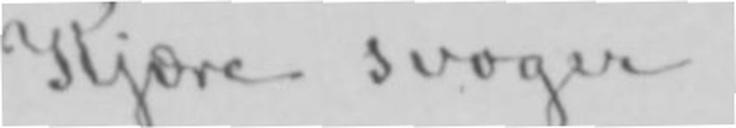Kjære svoger!
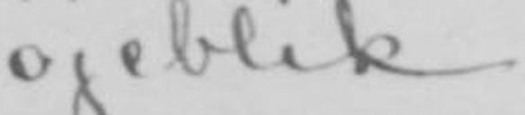øjeblik.
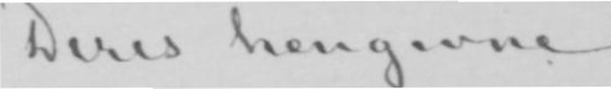Deres hengivne
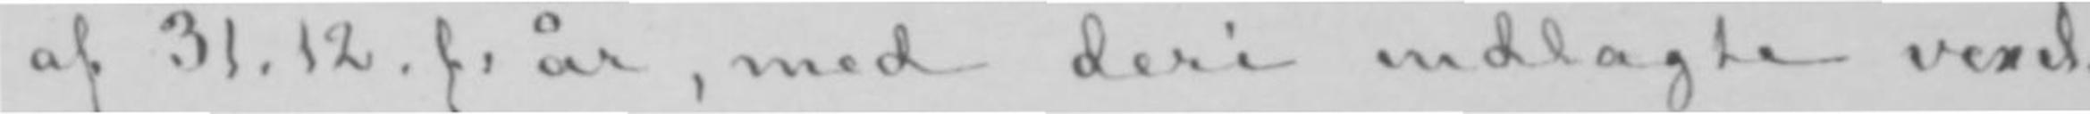af 31. 12. f: år, med deri indlagte vexel.
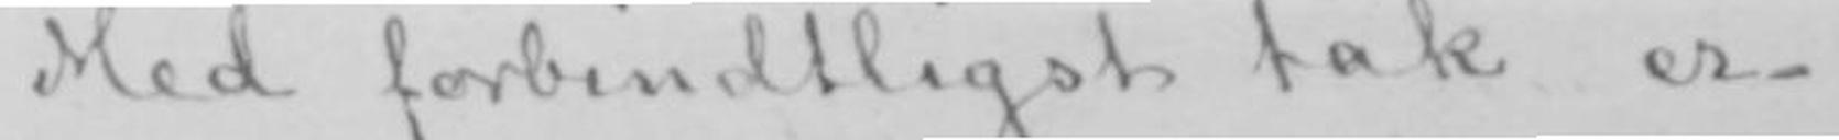Med forbindtligst tak er-
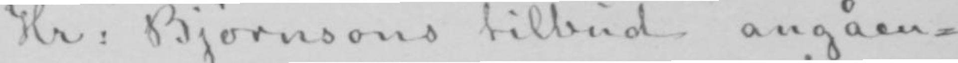Hr: Bjørnsons tilbud angåen-
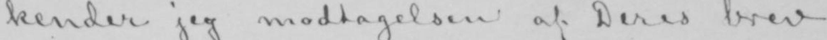kender jeg modtagelsen af Deres brev
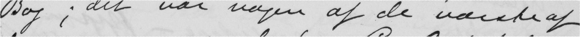Bog; det var nogen af de værste af
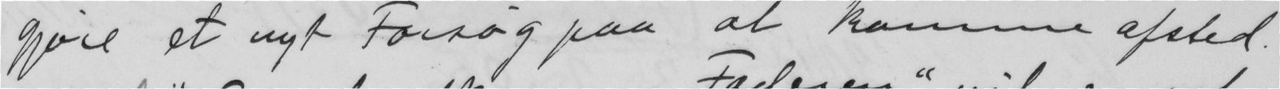gjøre et nyt Forsøg paa at komme afsted.
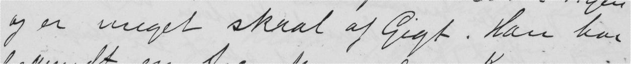og er meget skral af Gigt. Han har
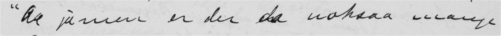"Aa jamen er der da noksaa mange
